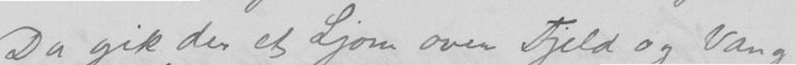Da gik der et Ljom over Fjeld og Vang
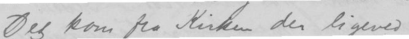Det kom fra Kirken der ligeved,
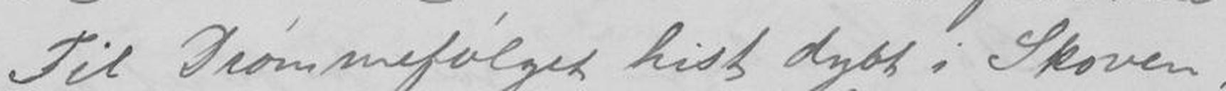Til Drømmefølget hist dybt i Skoven:
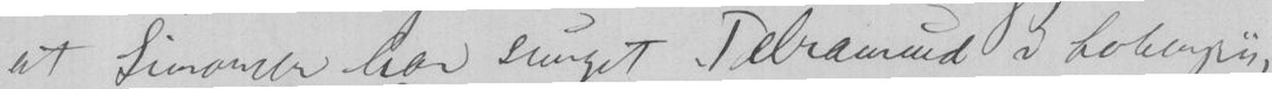at Simonsen har sunget Tebramund & Lohrengrin
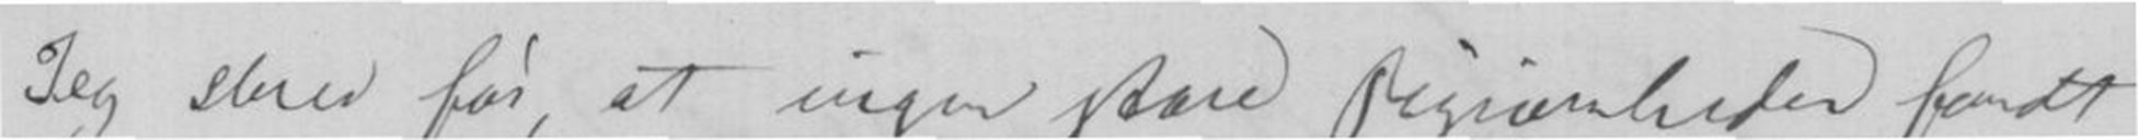Jeg skrev før, at ingen store Begivenheder fandt
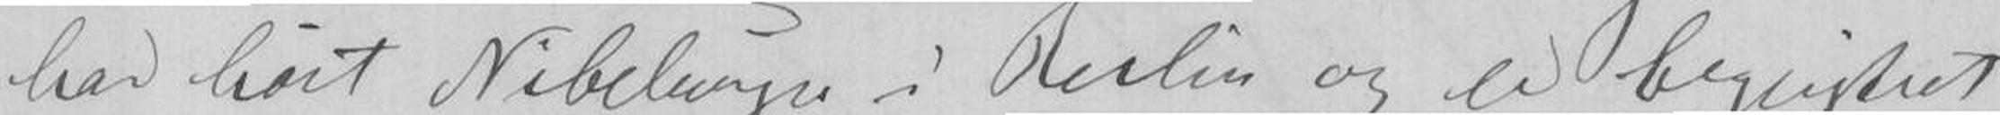har hørt Nibelunger i Berlin og er begeistret
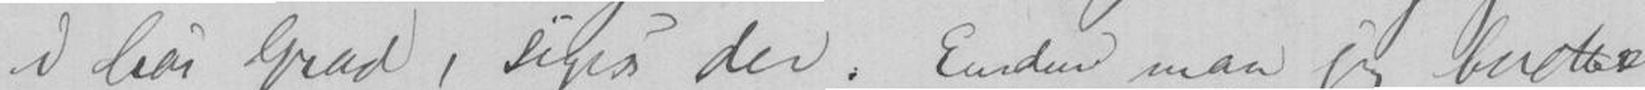i høi Grad, siges der. Endnu maa jeg berette
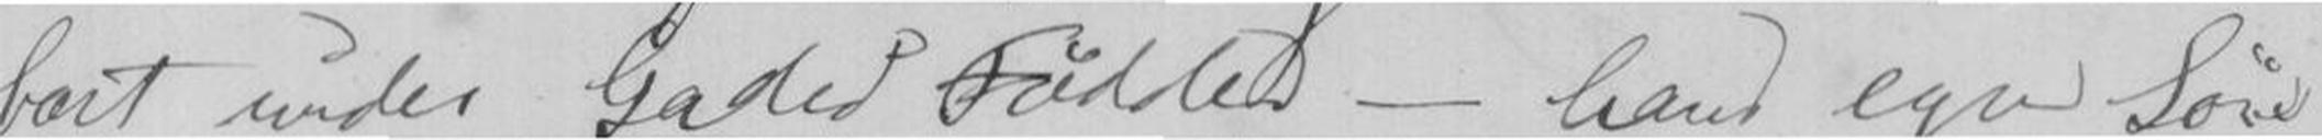bort under Gades Fødder - hans egen Søn
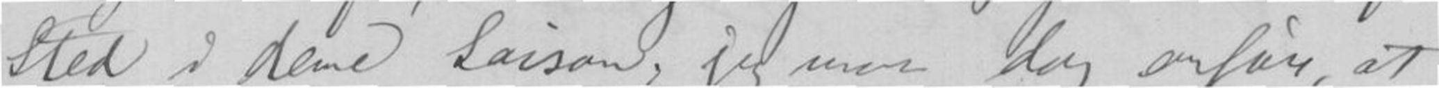Sted i denne Saison, jeg maa dog anføie, at
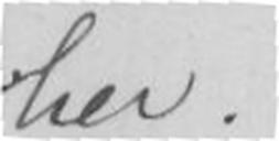her.
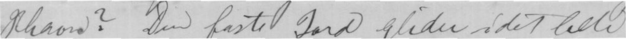Khavn? Den faste Jord glider idet lille
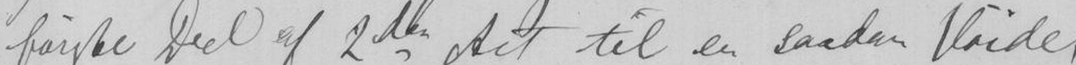første Deel af 2den Act til en saaden Høide,
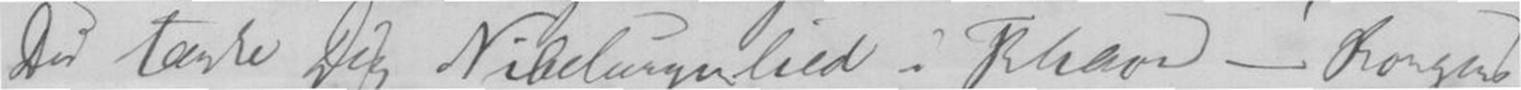Du tænke Dig Nibelungerlied i Khavn - Kongens
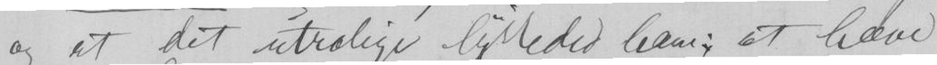og at det utrolige lykkedes ham; at hæve
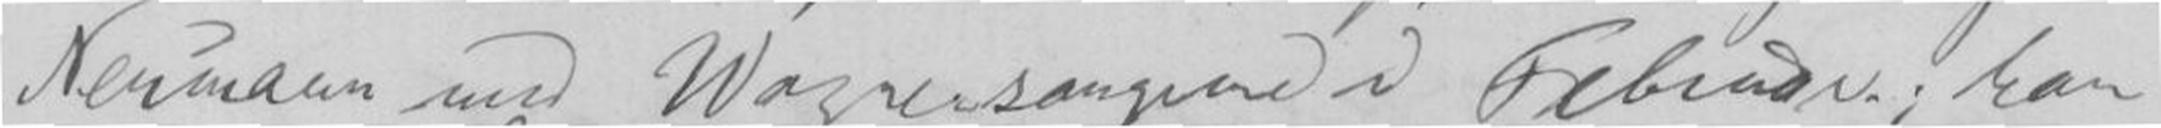Neumann med Wagnersangene i Februar, kan
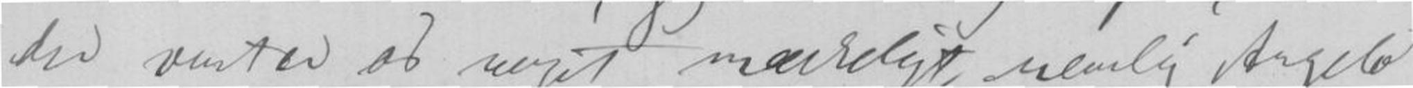der venter os meget mærkeligt, nemlig Angelo
